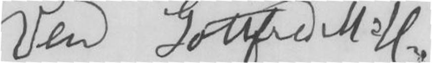Ven Gottfred M=H.
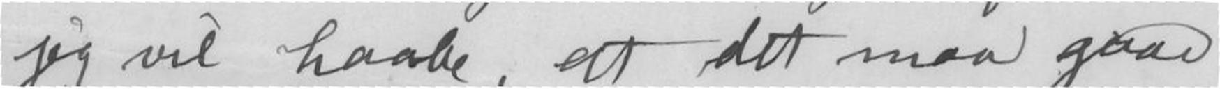jeg vil haabe at det maa gaae
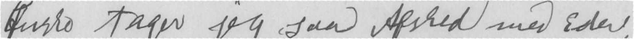Ønske tager jeg saa Afsked med Eder,
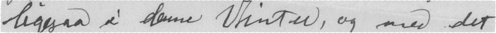ligesaa i denne Vinter, og med det
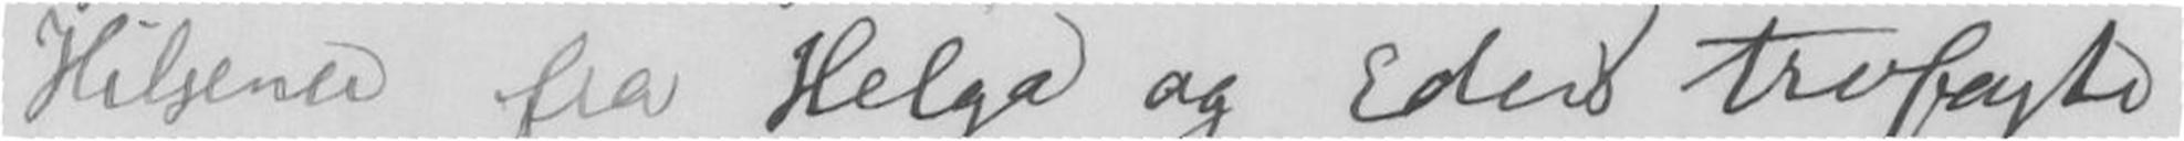Hilsener fra Helga og Eders trofaste
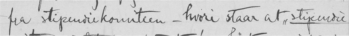fra stipendiekomiteen - hvori staar at "stipendie
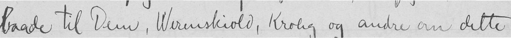baade til Dem, Werenskiold, Krohg og andre om dette
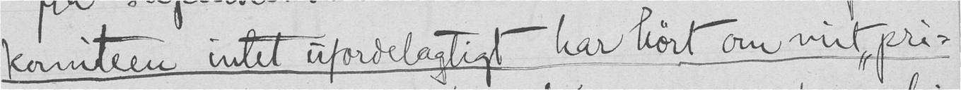komiteen intet ufordelagtigt har hørt om mit "pri-
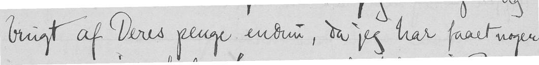brugt af Deres penge endnu, da jeg har faaet nogen
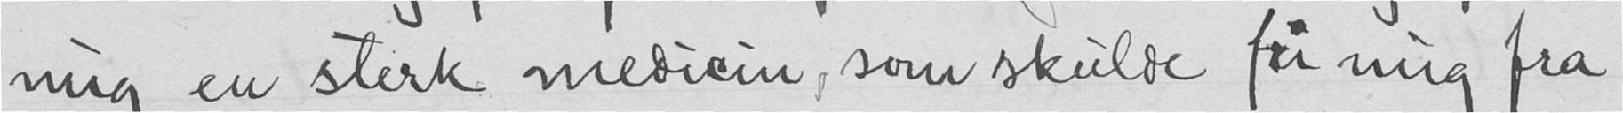mig en sterk medicin, som skulde få mig fra
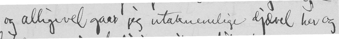og alligivel gaar jeg utaknemlige djævel her og
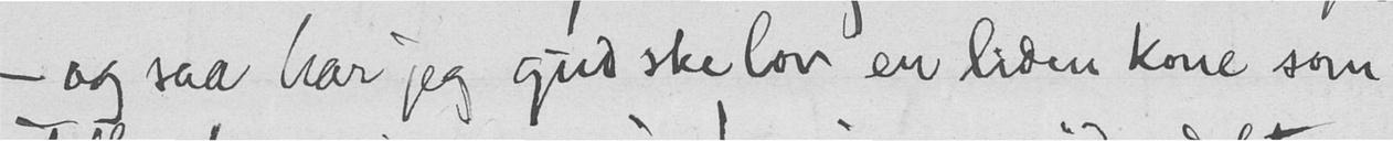- og saa har jeg gud ske lov en liden kone som
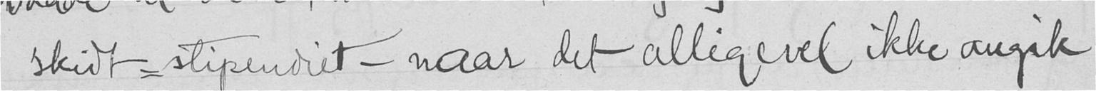skidt-stipendet - naar det alligevel ikke angik
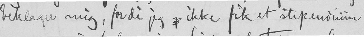beklager mig, fordi jeg  ikke fik et stipendium
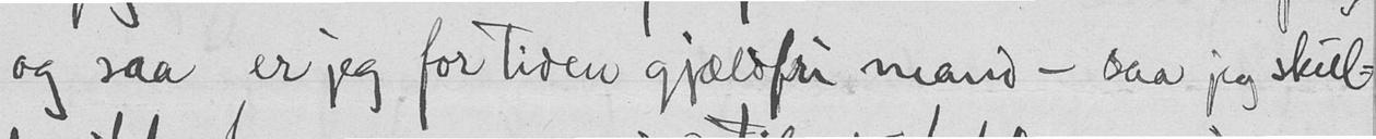og saa er jeg for tiden gjældfri mand - saa jeg skuld-
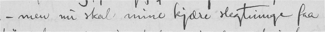- men nu skal mine kjære slegtninge faa
